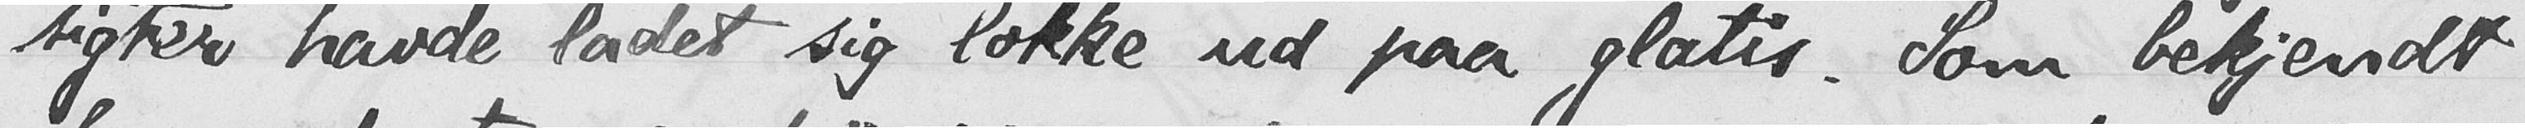sigter havde ladet sig lokke ud paa glatis. Som bekjendt
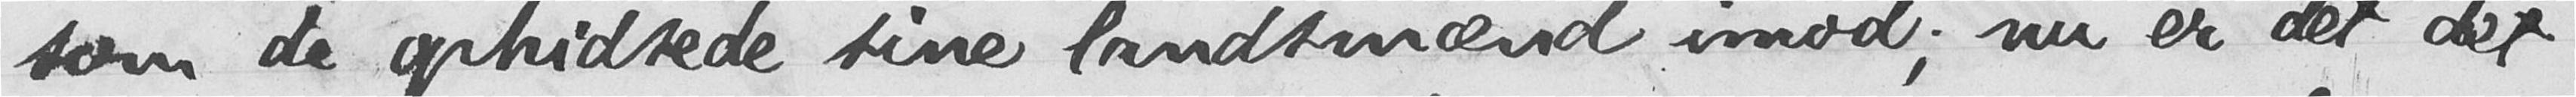som de ophidsede sine landsmænd imod; nu er det det
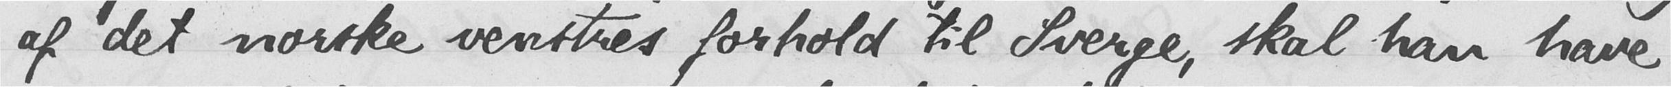af det norske venstres forhold til Sverge, skal han have
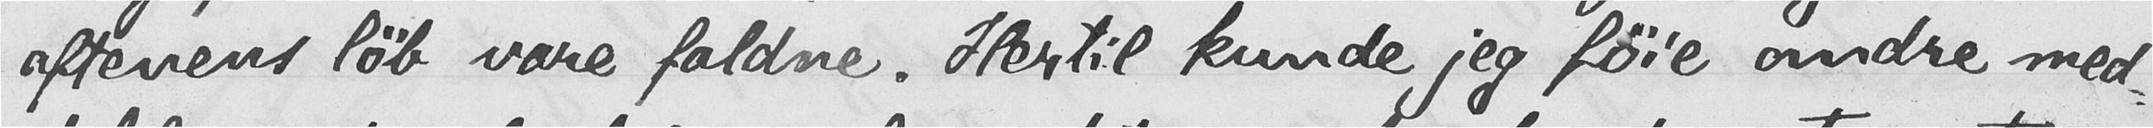aftenens løb vare faldne. Hertil kunde jeg føie andre med-
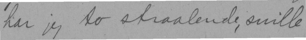har jeg to straalende, snille
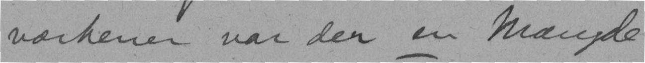værkener var der en Mængde
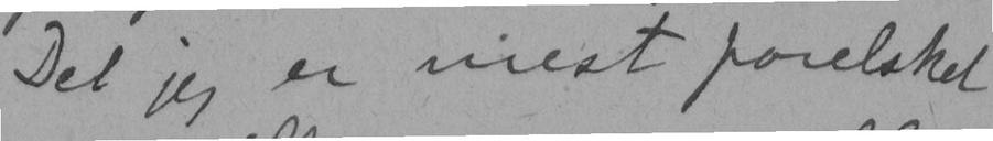Del jeg er mest forelsket
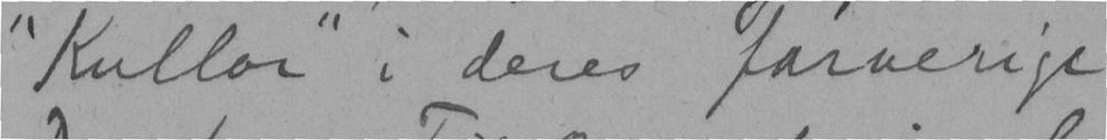"Kullor" i deres farverige
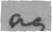og
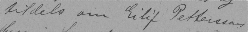tildels om Eilif Petterssens
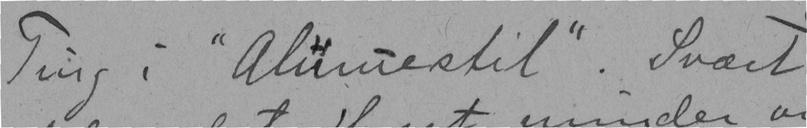Ting i "Almuestil". Svært
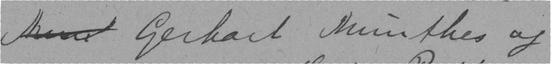Gerhart Munthes og
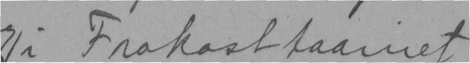i Frokosttaarnet
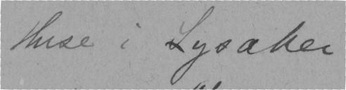Huse i Lysaker
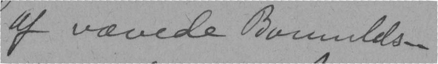af vævede Bomulds-
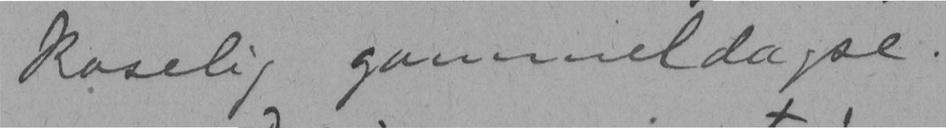koselig gammeldagse.
